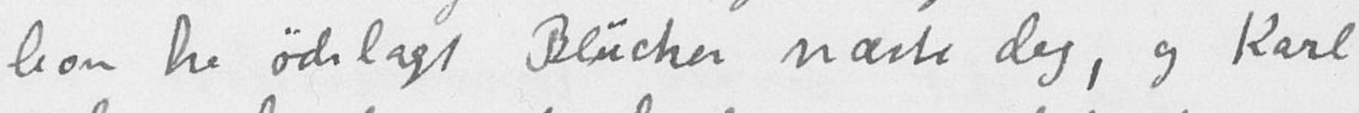leon ha ødelagt Blücher næste dag, og Karl
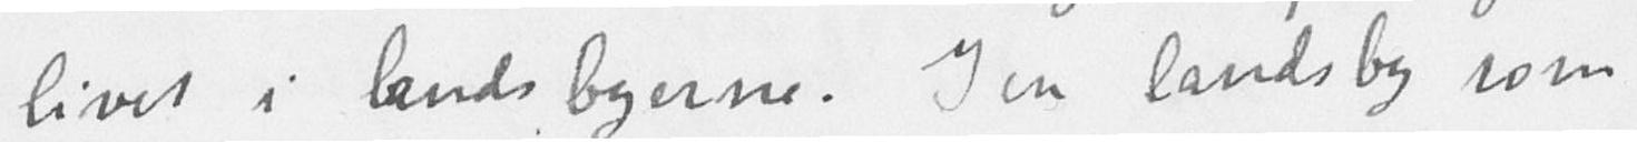livet i landsbyerne. I en landsby som
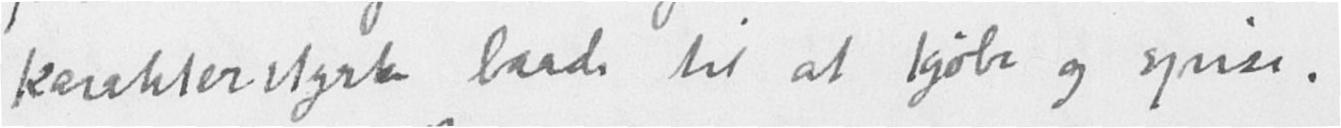karakterstyrke baade til at kjøbe og spise.
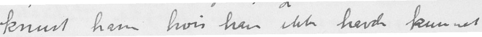knust ham hvis han ikke havde kunnet
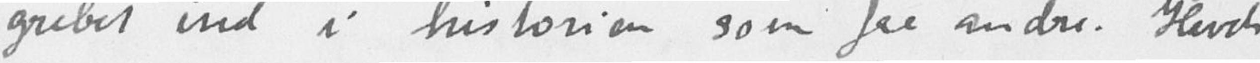grebet ind i historien som faa andre. Havde
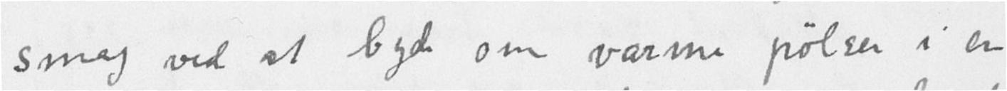smag ved at byde om varme pølser i en
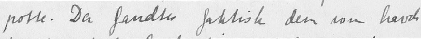potte. Der fandtes faktisk dem som havde
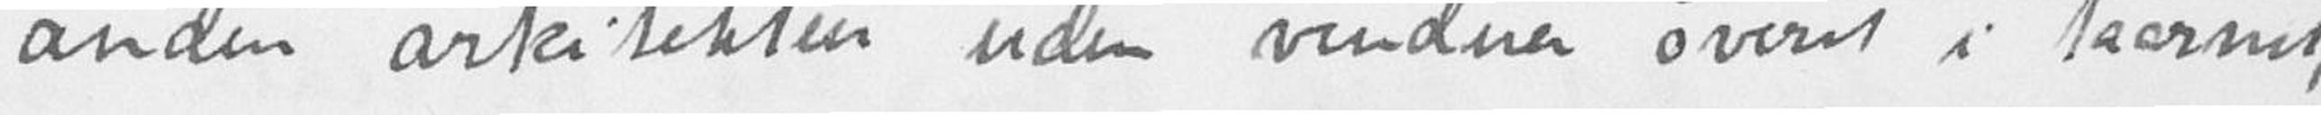anden arkitektur uden vinduer øverst i taarnet,
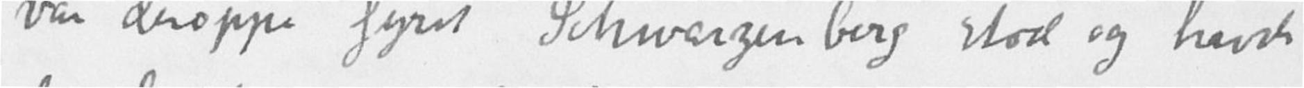var deroppe fyrst Schwarzenberg stod og havde
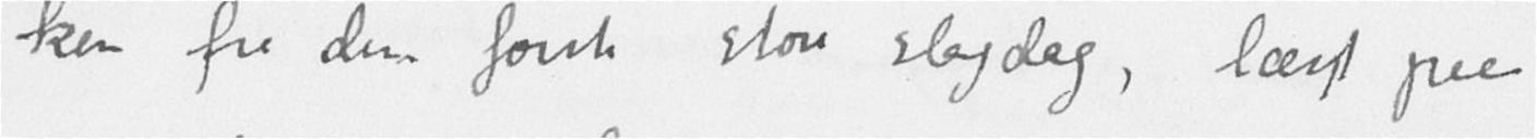ken fra den første store slagdag, læst paa
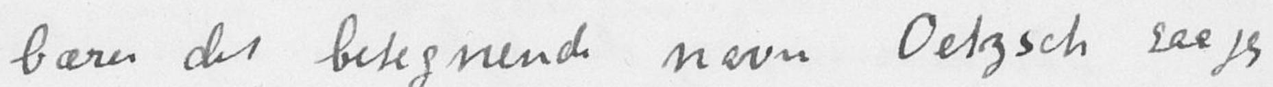bærer det betegnende navn Oetzsch saa jeg
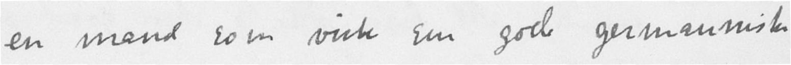en mand som viste sin gode germanniske
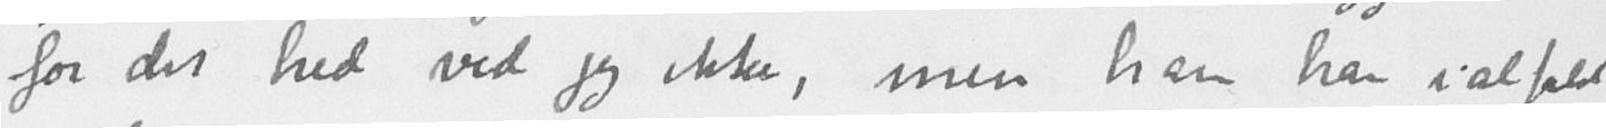for det hed ved jeg ikke, men han har i alfald
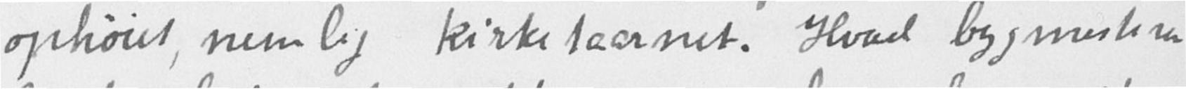ophøiet, nemlig kirketaarnet. Hvad bygmesteren
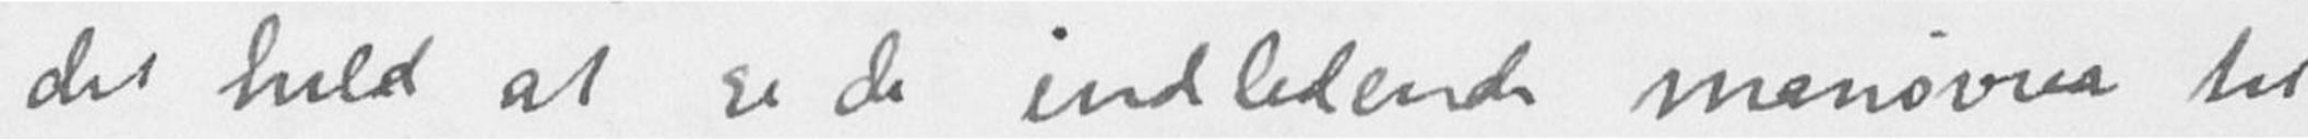det held at se de indledende manøvrer til
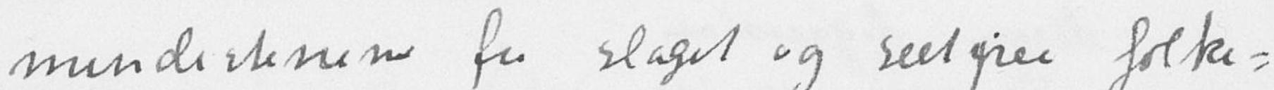mindestenene fra slaget og seet paa folke-
